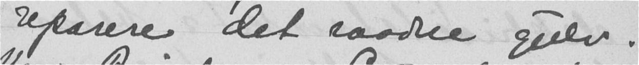reparere det raadne gulv.
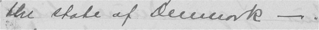the state of Denmark - .
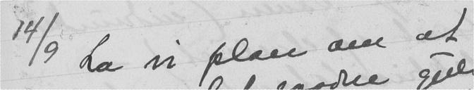14/9 La vi plan om at
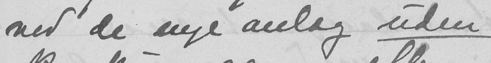ved de nye anlæg uden
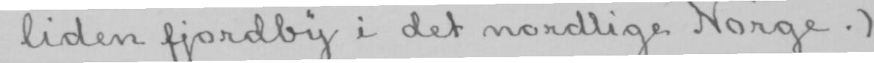liden fjordby i det nordlige Norge.)
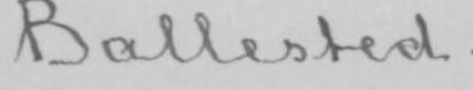Ballested.
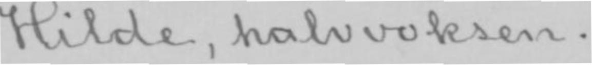Hilde, halvvoksen.
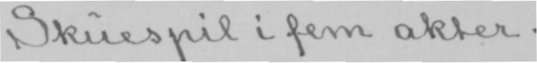Skuespil i fem akter.
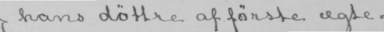hans døttre af første ægte-
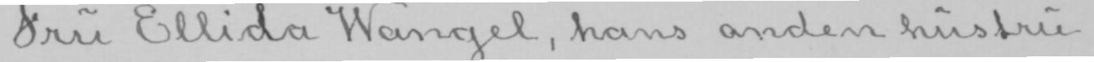Fru Ellida Wangel, hans anden hustru.
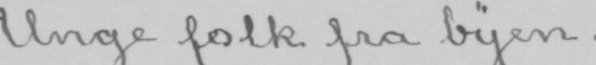Unge folk fra byen.
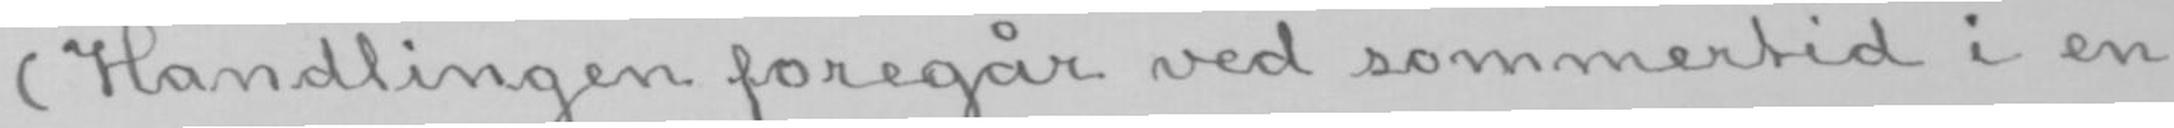(Handlingen foregår ved sommertid i en
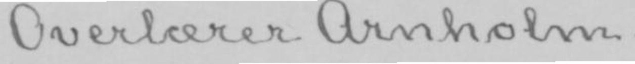Overlærer Arnholm.
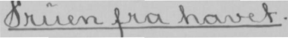Fruen fra havet.
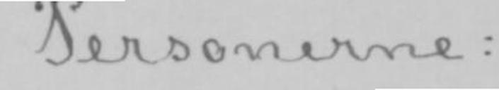Personerne:
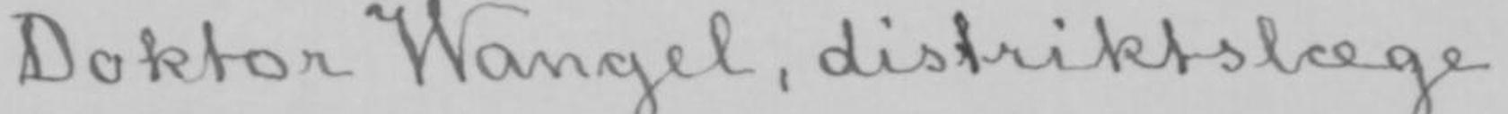Doktor Wangel, distriktslæge.
